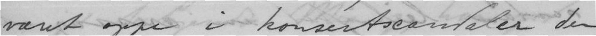været oppe i konsertscandaler der
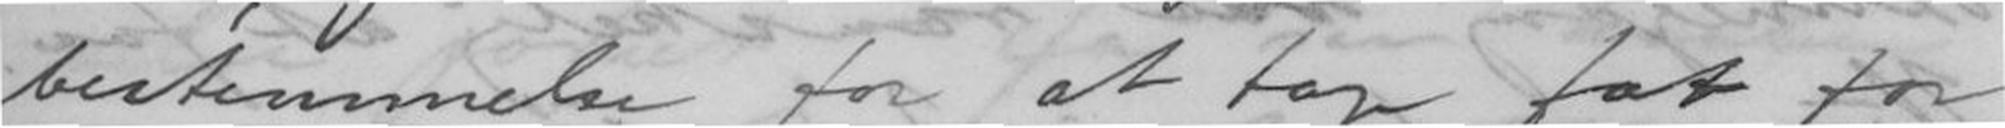bestemmelse for at tage fat for
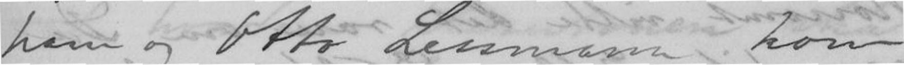ham og Otto Lessmann kom
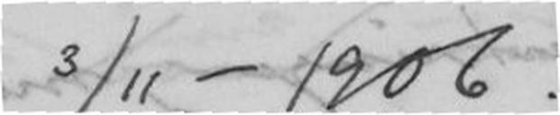3/11-1906
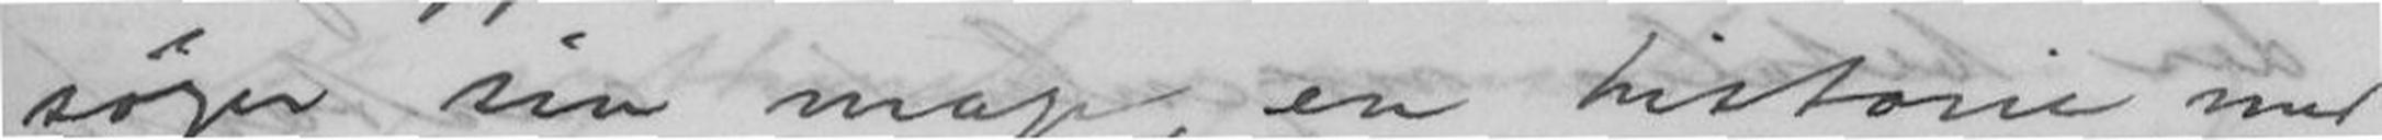søger sin mage, en historie med
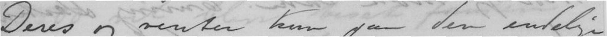Deres, og venter kun paa den endelige
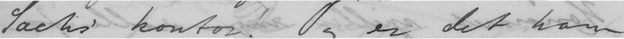Sachs' kontor! Og er det ham
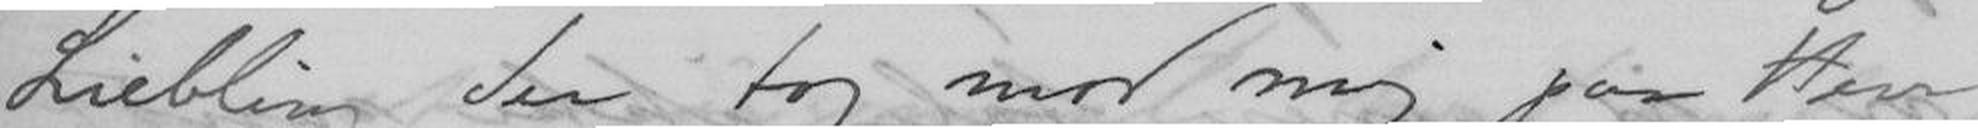Liebling der tog mod mig paa Herr
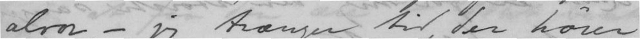alvor, - jeg trænger tid, der hører
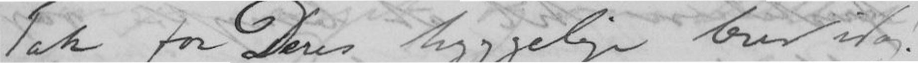Tak for Deres hyggelige brev idag!
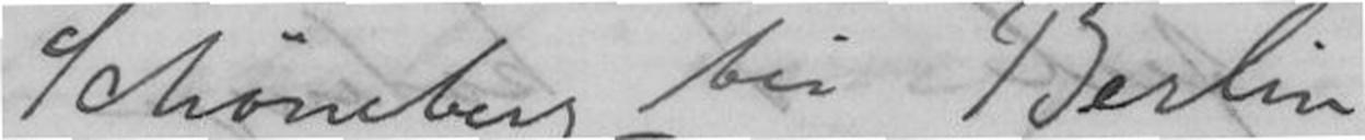Schöneberg bei Berlin
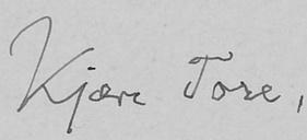Kjære Tore,
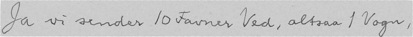Ja vi sender 10 Favner Ved, altsaa 1 Vogn,
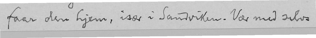faar den hjem, især i Sandviken. Vær med selv.
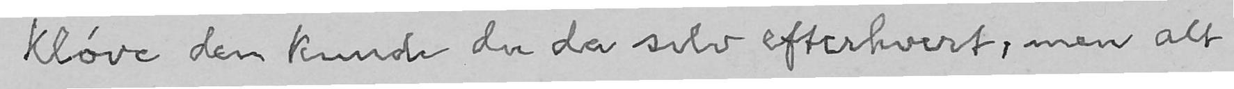kløve den kunde du da selv efterhvert, men alt
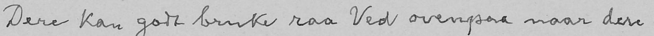Dere kan godt bruke raa Ved ovenpaa naar dere
